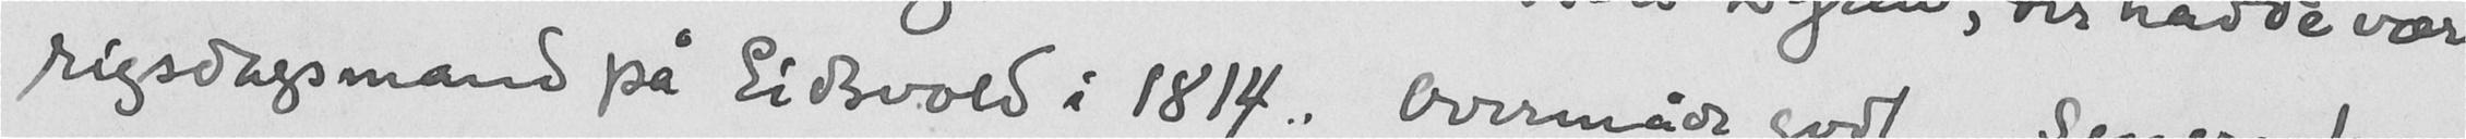rigsdagsmand på Eidsvold i 1814. Overmåde godt. Senere hos
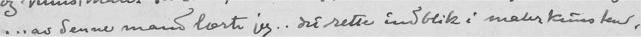...av denne mand lærte jeg.. det rette indblik i malerkunsten.
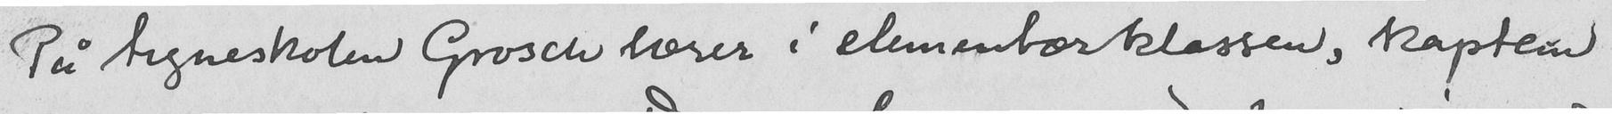På tegneskolen Grosch lærer i elementærklassen, kaptein
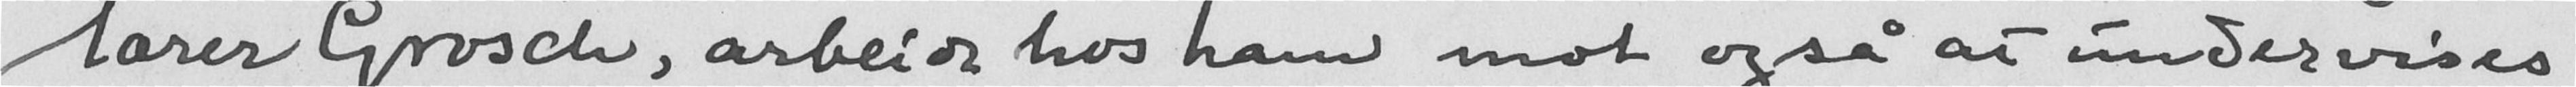lærer Grosch, arbeide hos ham mot også at undervises
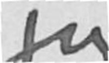jeg
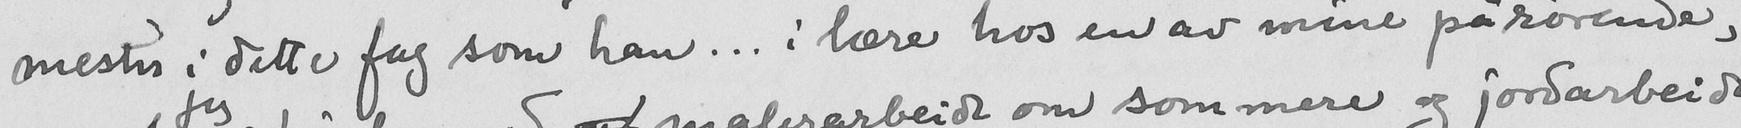mester i dette fag som han... i lære hos en av mine pårørende,
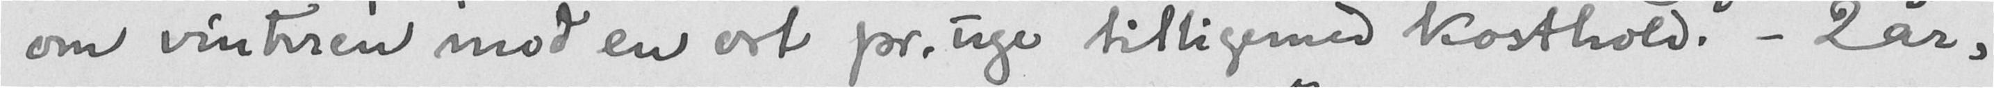om vinteren mod en ort pr. uge tilligemed kosthold. - 2 år,
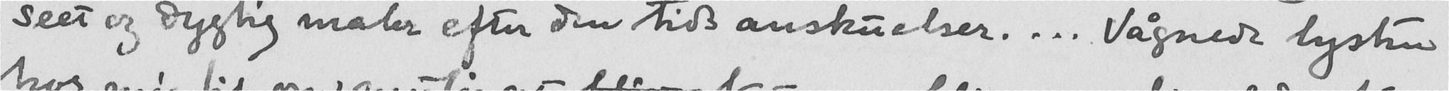seet og dygtig maler efter den tids anskuelser... Vågnede lysten
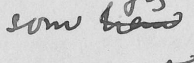som han
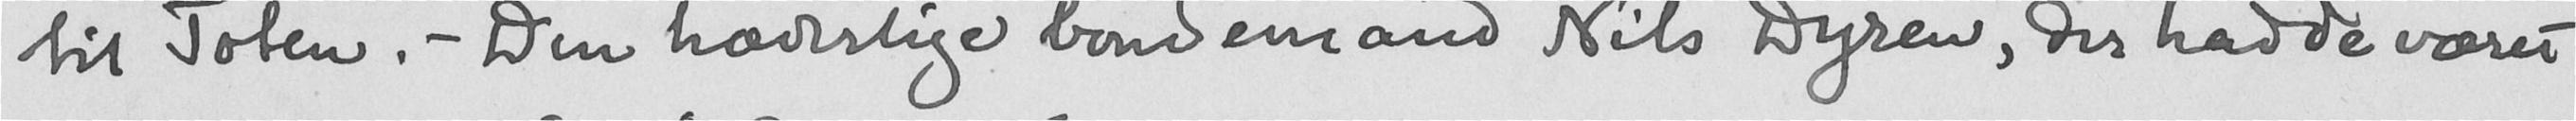til Toten. - Den hæderlige bondemand Nils Dyren, der hadde været
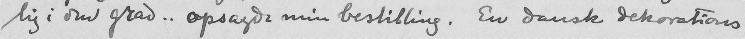lig i den grad.. opsagde min bestilling. En dansk dekorations
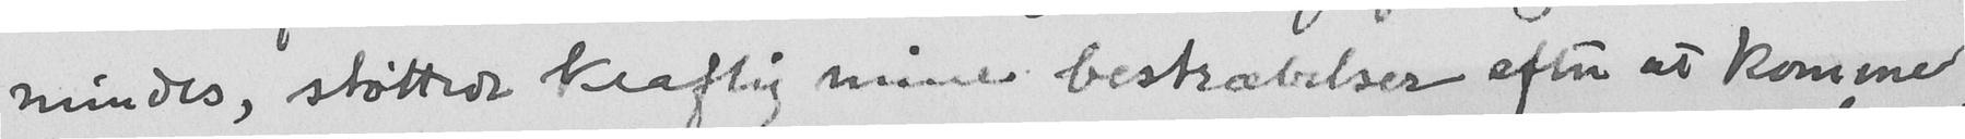mindes, støttede kraftig mine bestræbelser efter at komme
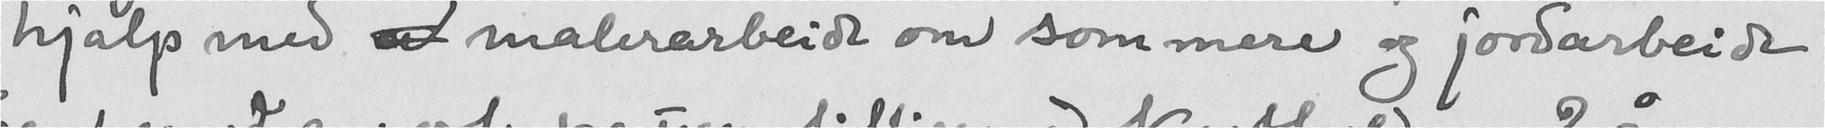hjalp med a malerarbeide om sommere og jordarbeide
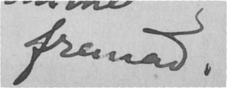fremad.
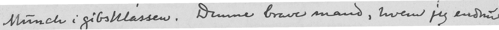Munch i gipsklassen. Denne brave mand, hvem jeg endnu
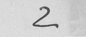2
